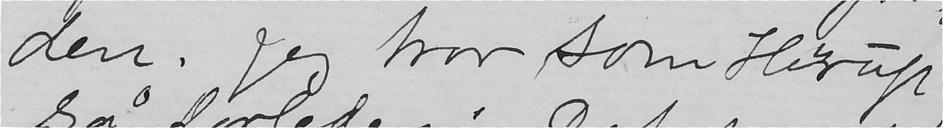den. Jeg tror som Hørup
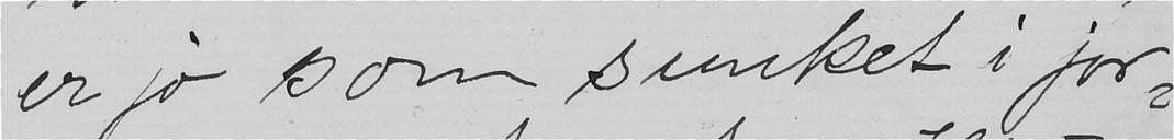er jo som sunket i jor-
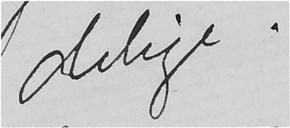delige.
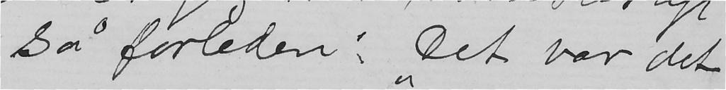så forleden: "Det var det
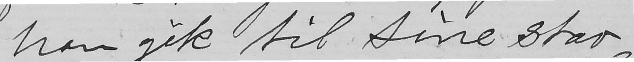han gik til sine stæv-
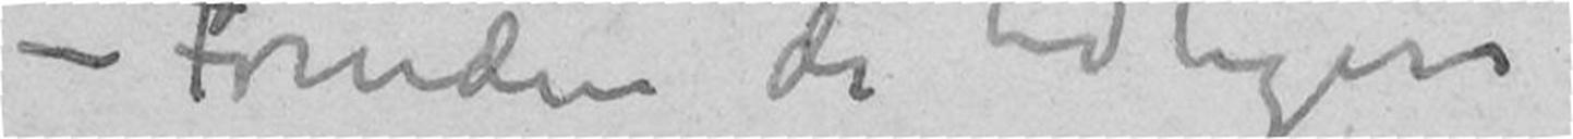– Foruden de tidligere
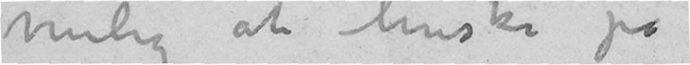venlig at huske på
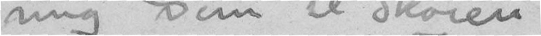mig dem til Skøien
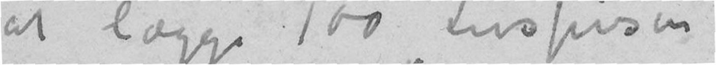at lægge 100 «Livsfrisen»
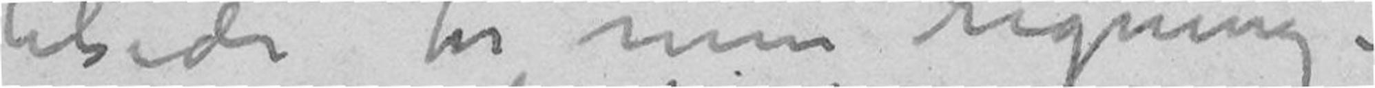tilside for min regning –
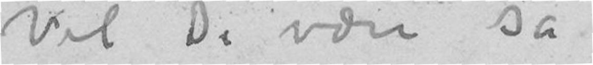Vil De være så
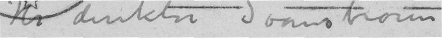Hr direktør Svanström
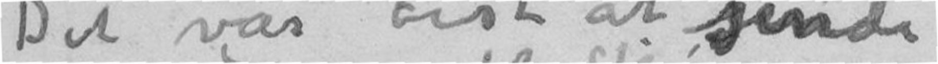Det var best at sende
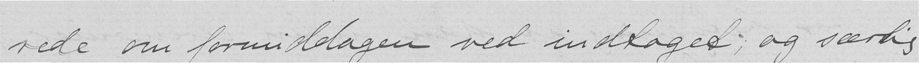rede om formiddagen ved indtoget; og særlig
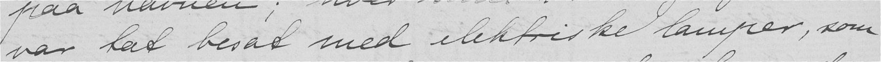var tæt besat med elektriske lamper, som
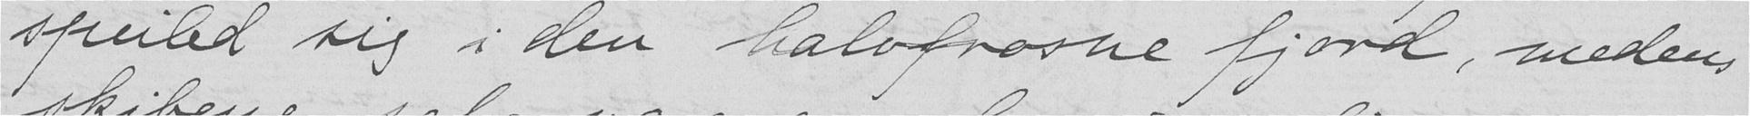speiled sig i den halvfrosne fjord, medens
